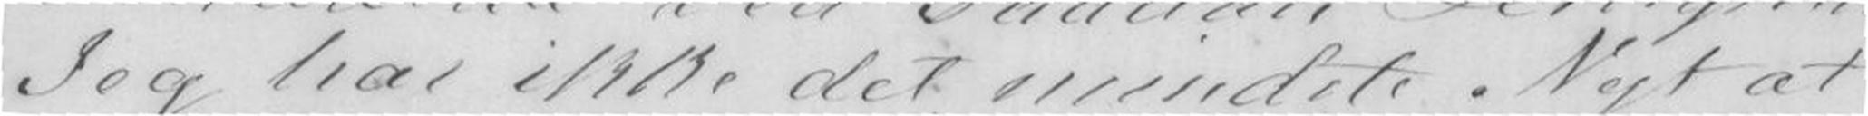Jeg har ikke det mindste Nyt at
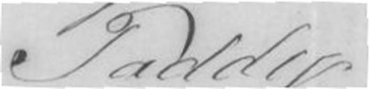Paddy
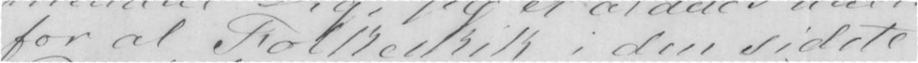for al Folkeskik i den sidste
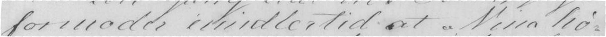formoder imidlertid at Nina hø-
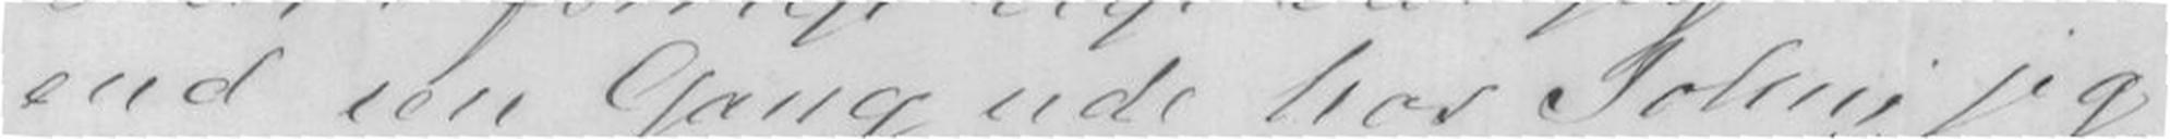end een Gang ude hos John jeg
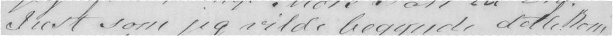Just som jeg vilde begynde dette kom
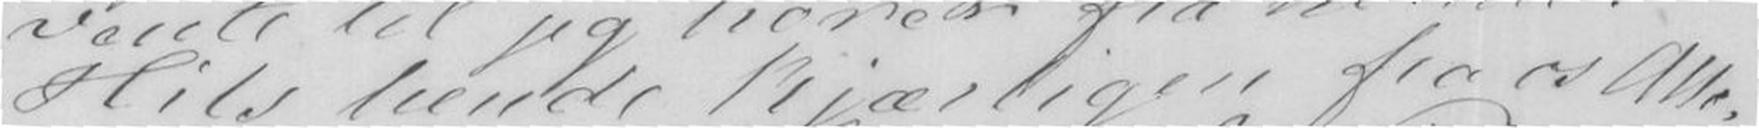Hils hende kjærligen fra os Alle,
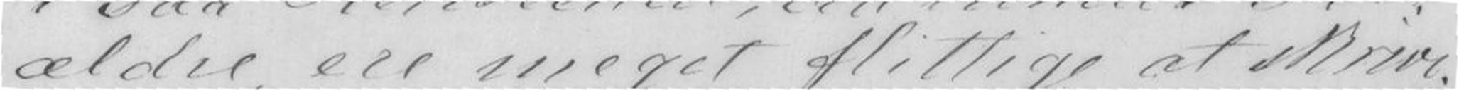ældre ere meget flittige at skrive.
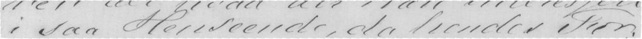i saa Henseende, da hendes For-
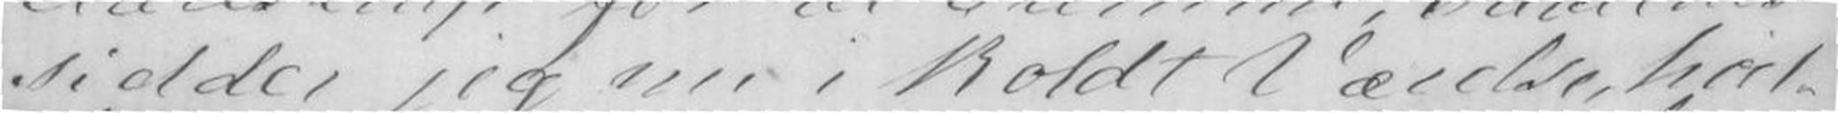sidder jeg nu i koldt Værelse, hvil-
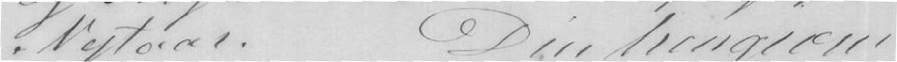Nytaar Din hengivne
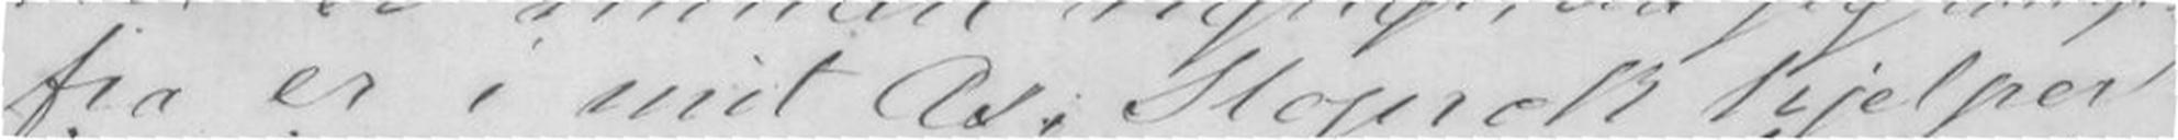fra er i mit Æs. Sloprok hjelper
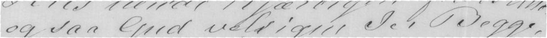og saa Gud velsigne Jer Begge,
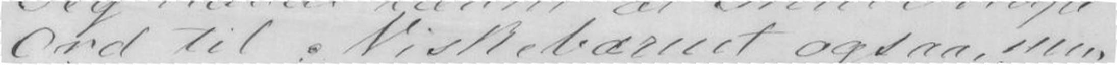Ord til Niskebarnet ogsaa, men
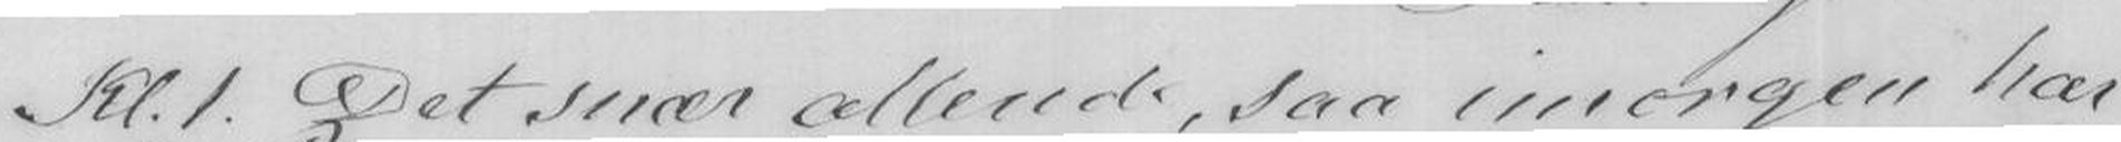Kl.1. Det snær allerede, saa imorgen har
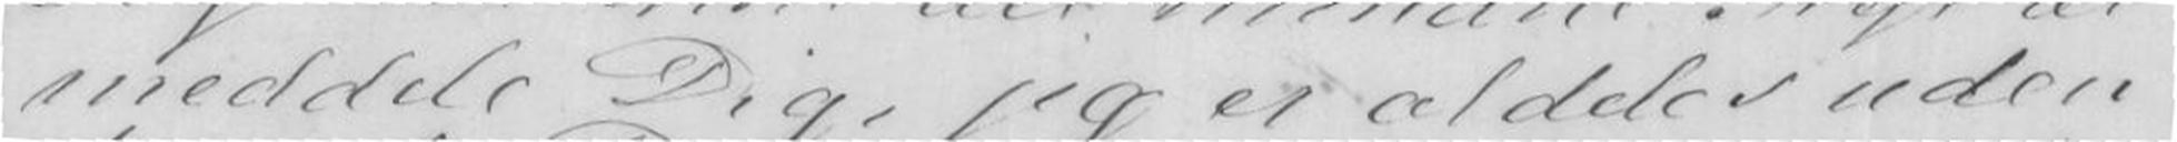meddele Dig, jeg er aldeles uden
